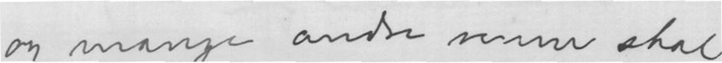og mange andre venner skal
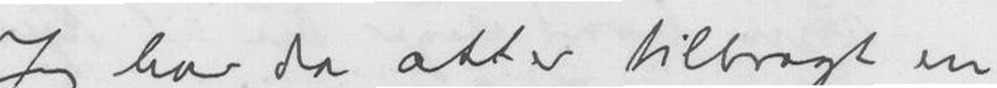Jeg har da atter tilbragt en
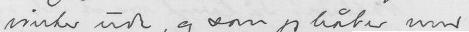vinter ude, og som jeg håber med
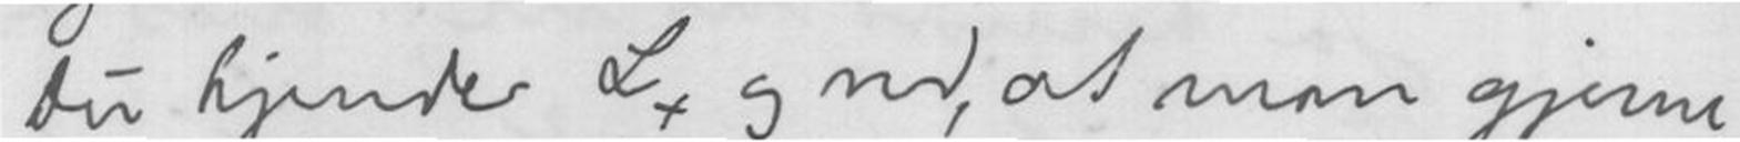Du kjender L, og ved, at man gjerne
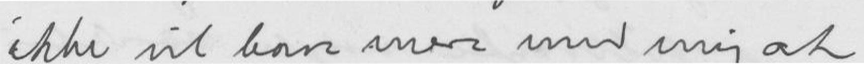ikke vil have mere med mig at
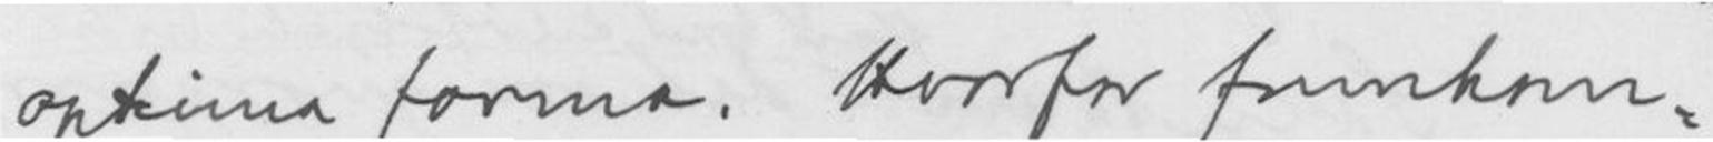optima forma. Hvorfor fremkom-
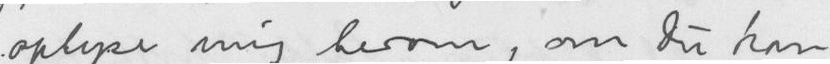oplyse mig herom, om du kan
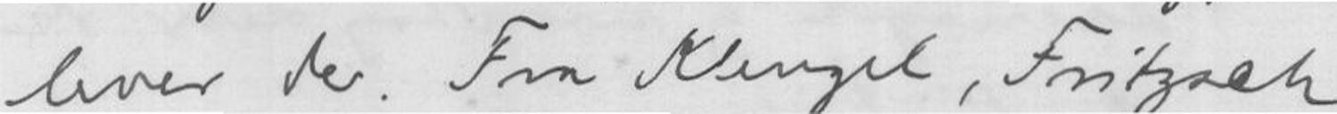lever der. Fra Klengel, Fritzsch
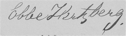Ebbe Hertzberg.
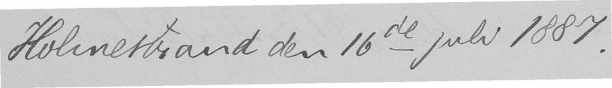Holmestrand den 16de juli 1887.
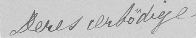Deres ærbødige
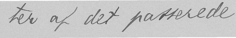ter af det passerede.
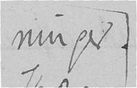ninger.
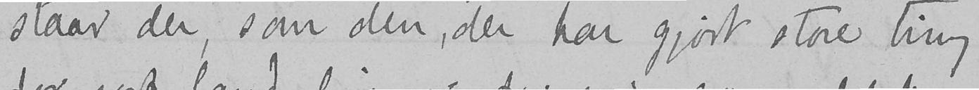staar der, som den, der har gjort store ting
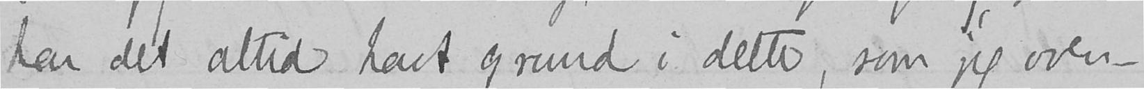har det altid havt grund i dette, som jeg oven-
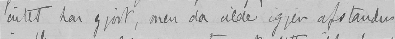intet har gjort, men da vilde igjen afstanden
